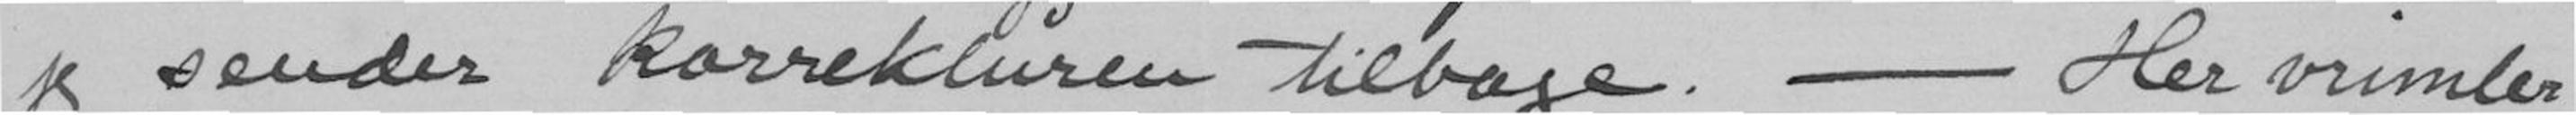jeg sender korrekturen tilbage. --- Her vrimler
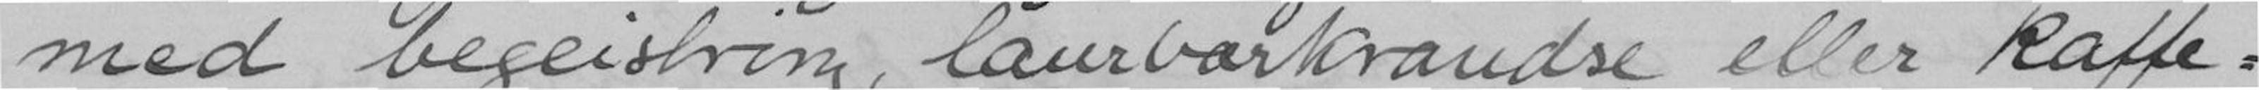med begeistring, laurbærkrandse eller kaffe-
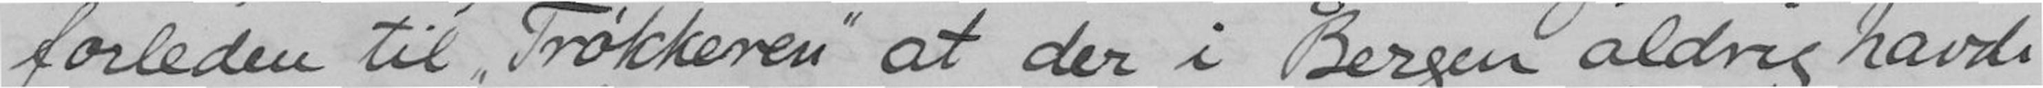forleden til Trøkkeren at der i Bergen aldrig havde
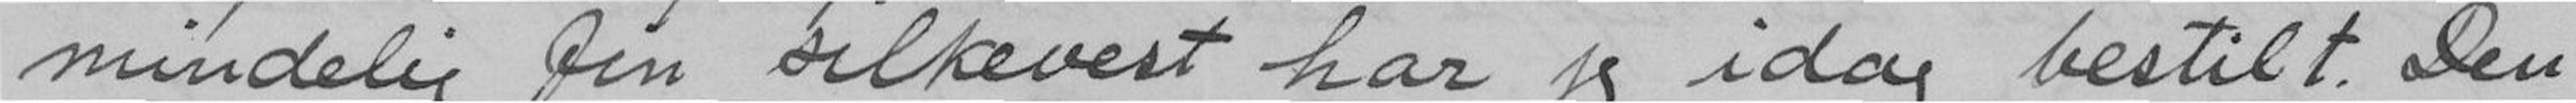mindelig fin silkevest har jeg idag bestlt. Den
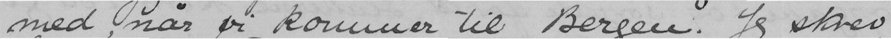med, når vi kommer til Bergen. Jeg skrev
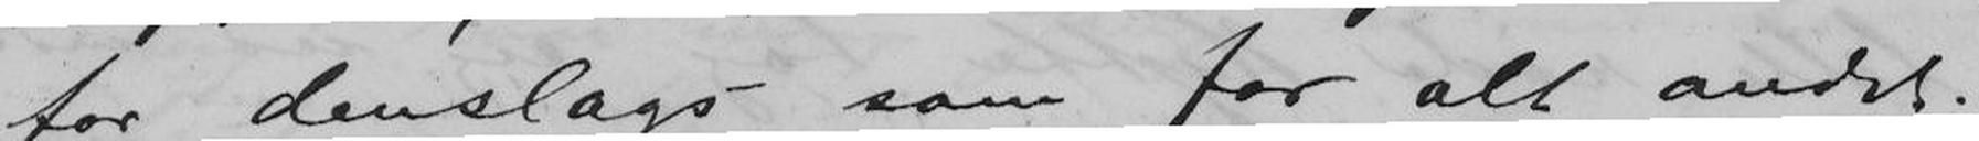for denslags som for alt andet.
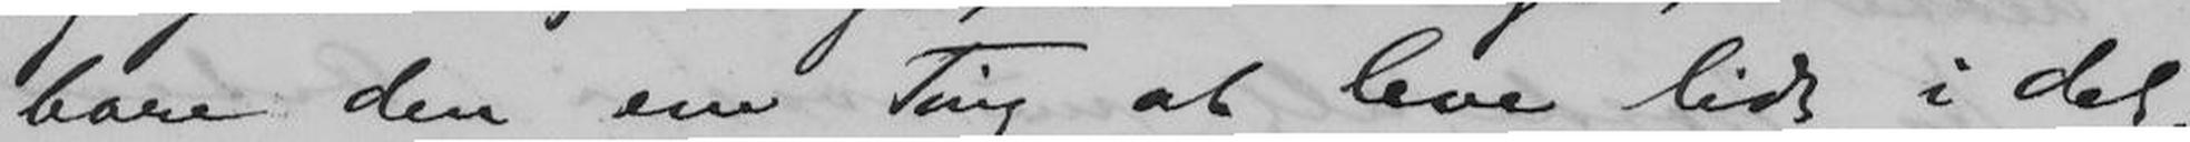bare den ene Ting at leve lidt i det,
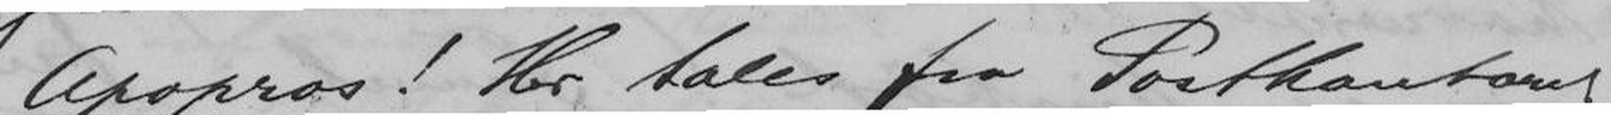Apropos! Her tales fra Postkontoret
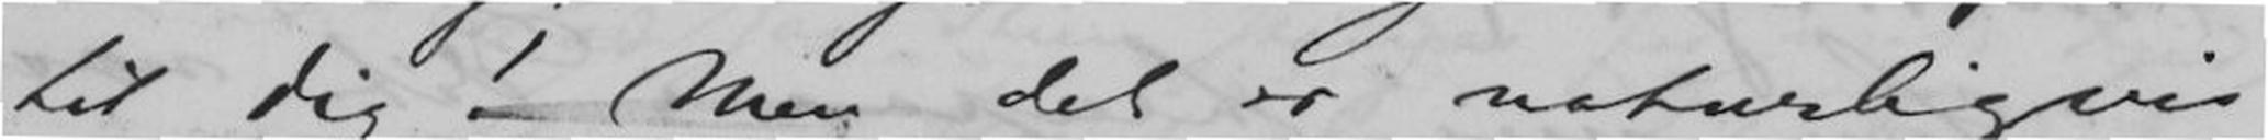til Dig! Men det er naturligvis
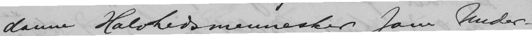danne Halvhedsmennesker som Under-
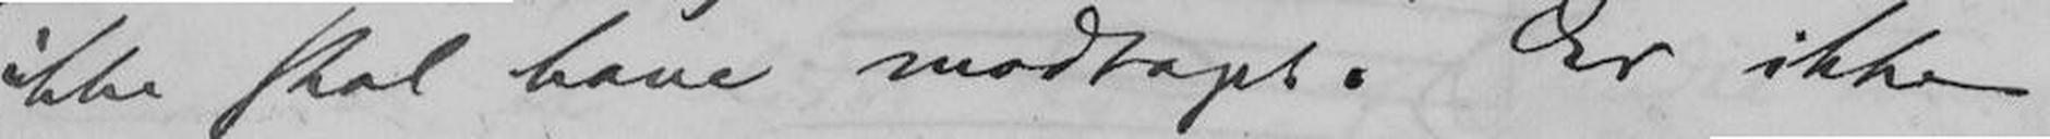ikke skal have modtaget. Er ikke
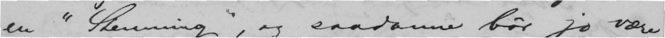en "Stemning", og saadanne bør jo være
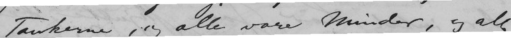Tankerne, og alle vore Minder, og alt
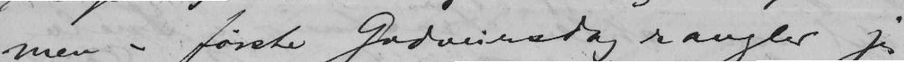men - første Godveirsdag rangler jeg
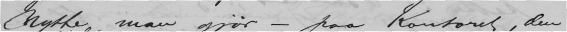Nytte, man gjør - paa Kontoret, den
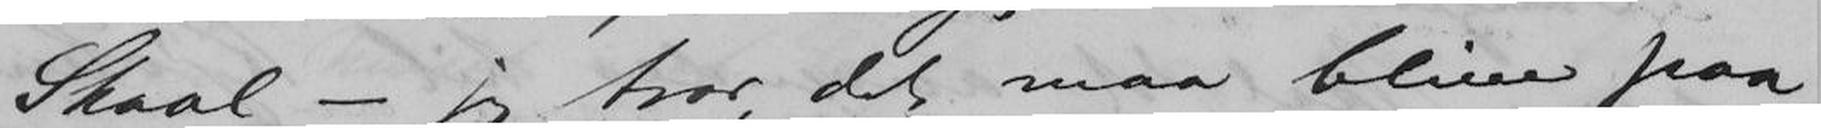Skaal - jeg tror, det maa blive paa
